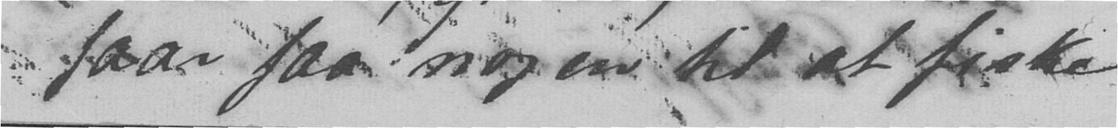faar faa nogen til at fiske
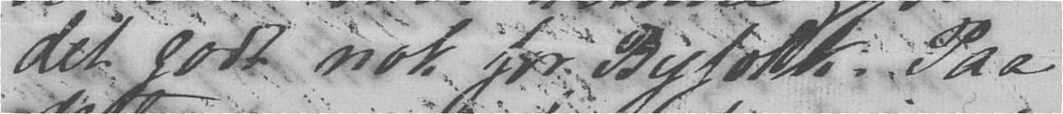det godt nok for Byfolk. Paa
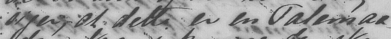siger at dette er en Talemaa-
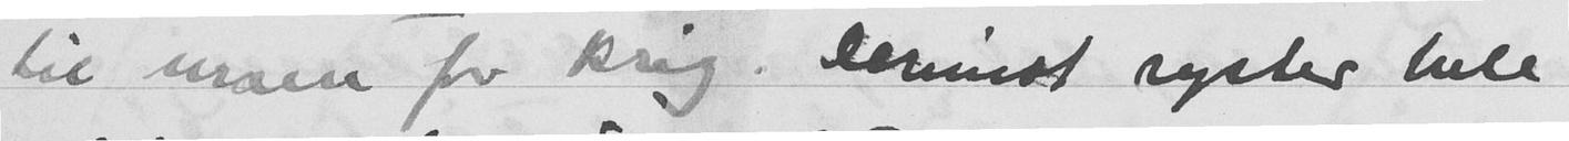til uroen for krig. Derimot ryster hele
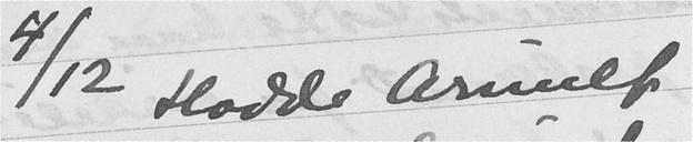4/12 Hadde Arnulf
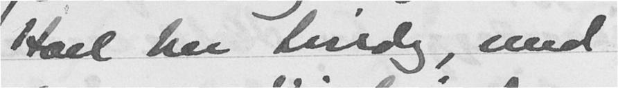Hoel her tirsdag, med
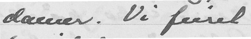damer. Vi feiret
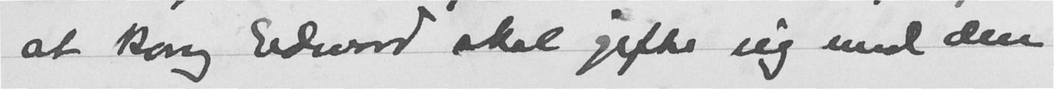at kong Edvard skal gifte sig med den
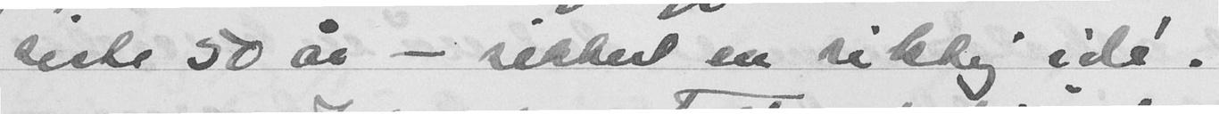siste 50 år - sikkert en riktig idé.
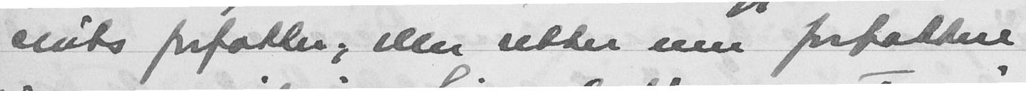snits forfatter; eller setter enn fortattere
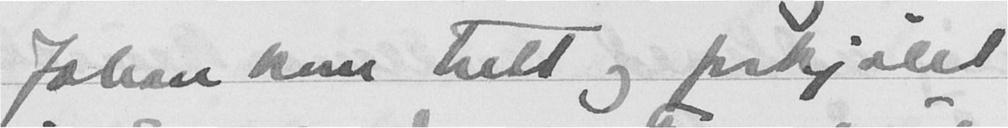Johan kom trett og forkjølet
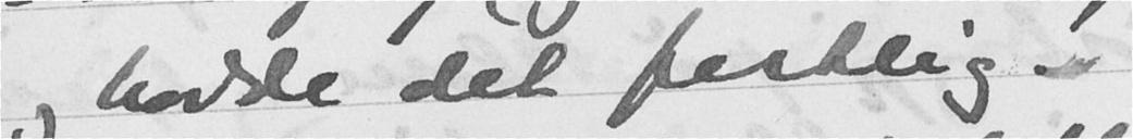og hadde det festlig!
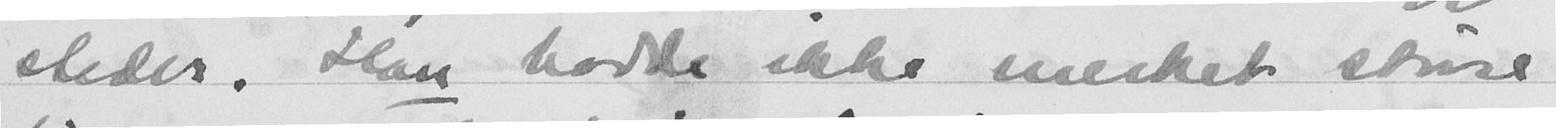steder. Han hadde ikke merket større
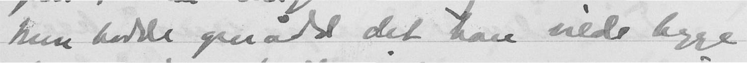Han hadde opnådd det han vilde begge
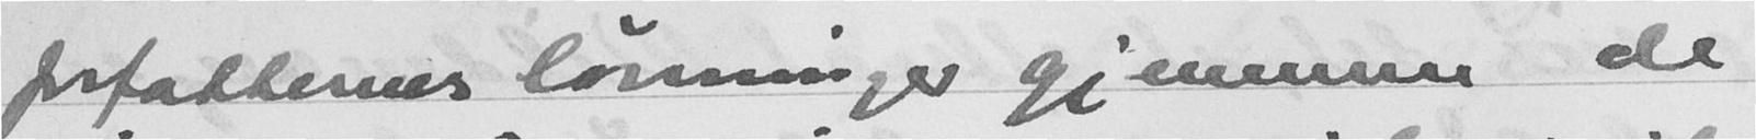forfatternes lønninger gjennom de
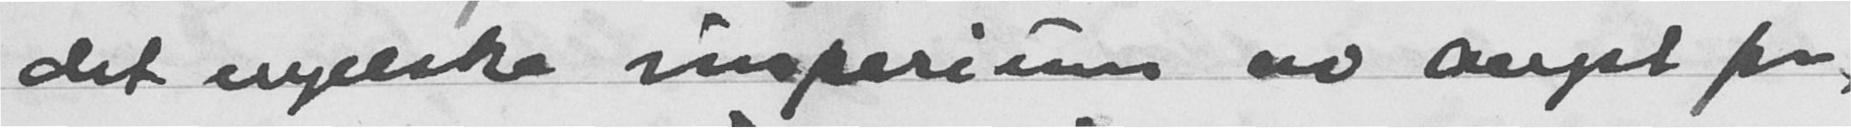det engelske imperium av angst for,
